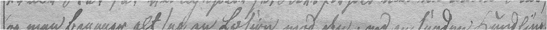og man begaaer altsaa en Læsion mod den, med en saadan Handling.
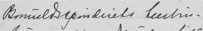Bomuldsspinderets turbin-
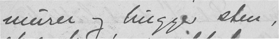murer og hugger sten,
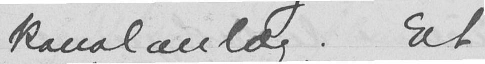kanalanlæg. Et
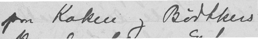paa Kaken og Bødtkers
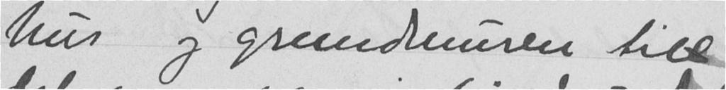hus og grundmuren til
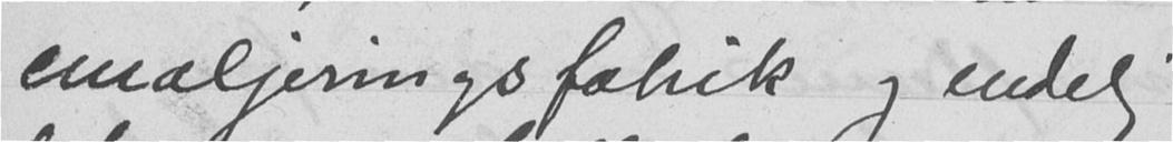emaljeringsfabrik og endelig
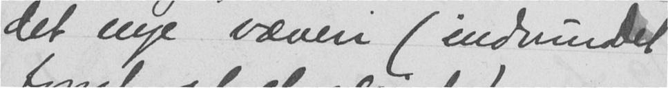det nye væveri (indvundet
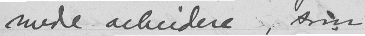mede arbeidere, som
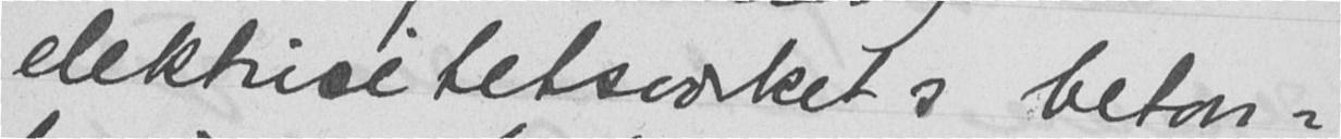elektrisitetsværkets beton-
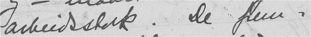arbeidsstok. De frem-
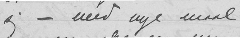sig - med nye maal
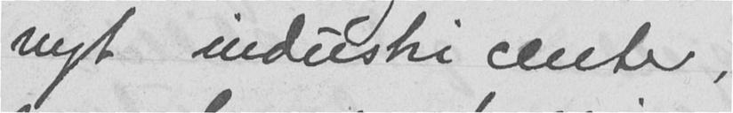nyt industricenter,
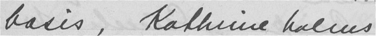basis, Kathrine holms
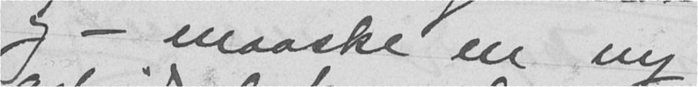og - maaske en ny
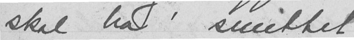skal ha' smittet
